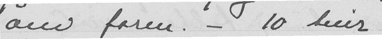om form. - 10 tiur
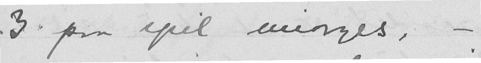3 paa spil imorges, -
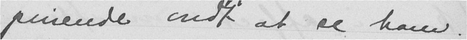pinende ondt at se ham.
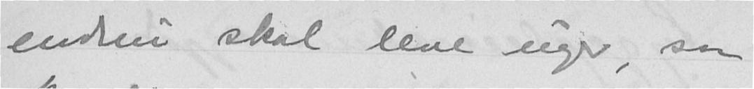endnu skal leve uger, saa
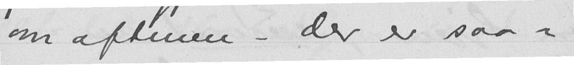om aftenen - der er saa-
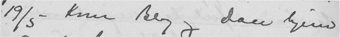19/5 Kom Berg og Don hjem
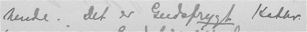hende. Det er Gudsfrygt Kathr.
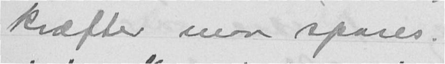kræfter maa spares.
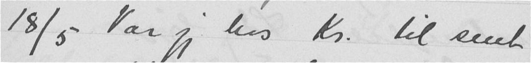18/5 Var jeg hos Kr. til sent
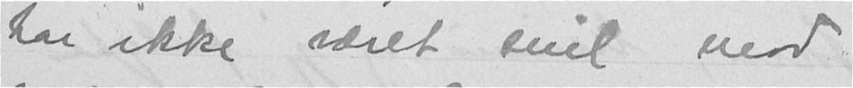har ikke været snil mod
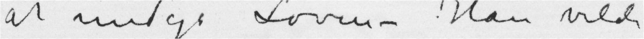at undgå Loven – Han vilde
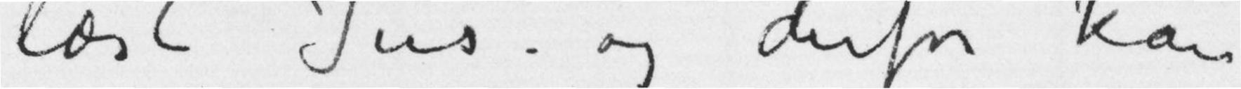læst Jus og derfor kan
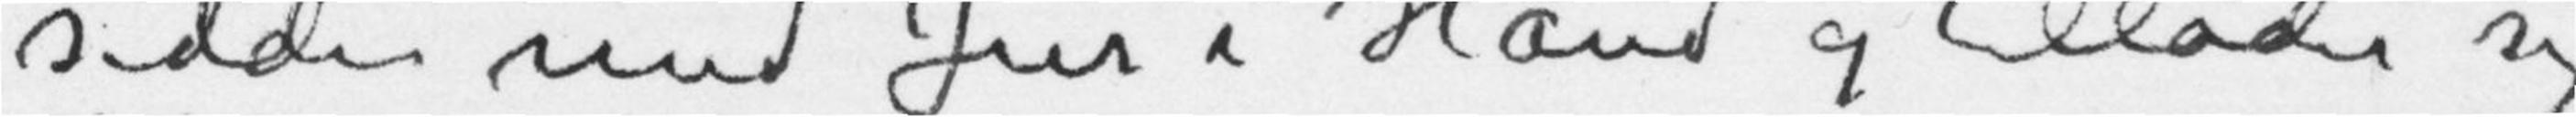sidder med Jus i Hånd og tillader sig
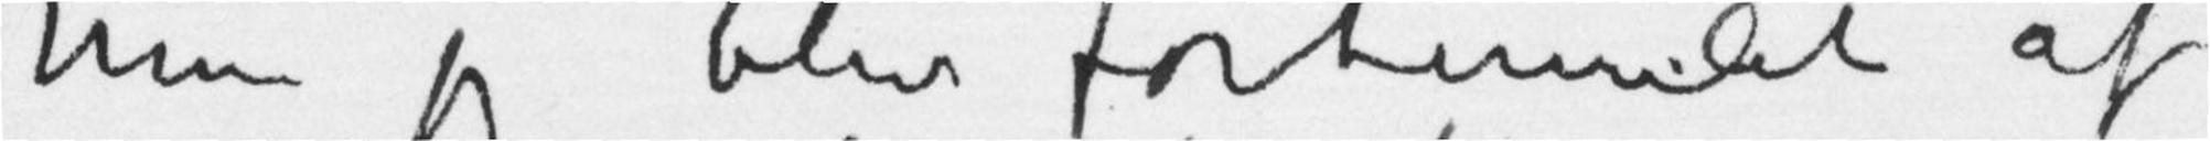Men jeg blev fortumlet af
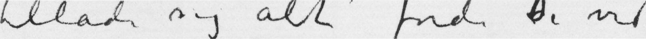tillade sig alt fordi Dde ved
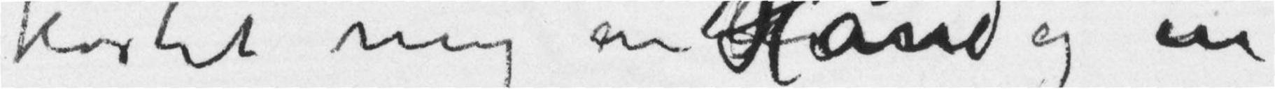kostet mig en Hånd og en
